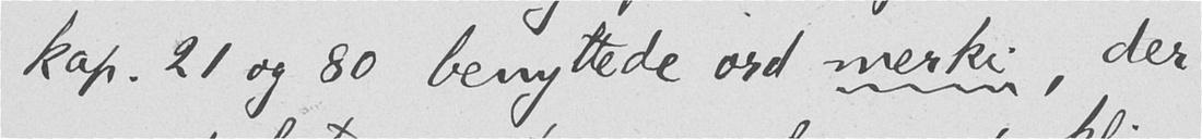kap. 21 og 80 benyttede ord merki, der
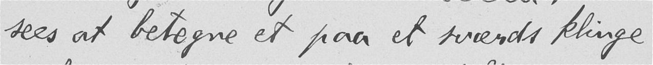sees at betegne et paa et sværds klinge
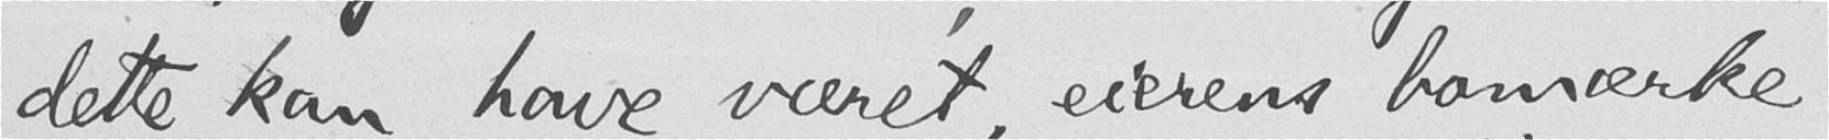dette kan have været, eierens bomærke
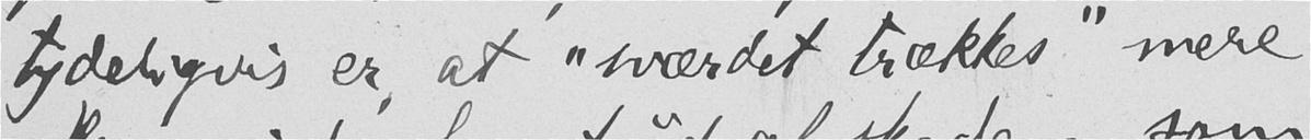tydeligvis er, at "sværdet trækkes" mere
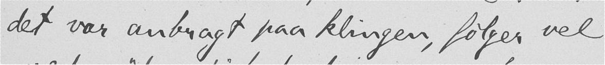det var anbragt paa klingen, følger vel
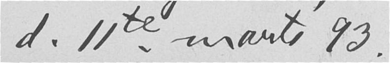d. 11te marts 93.
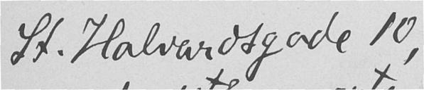St. Halvardsgade 10,
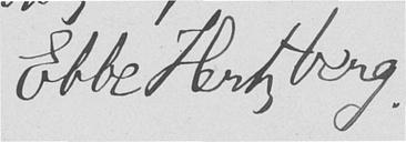Ebbe Hertzberg.
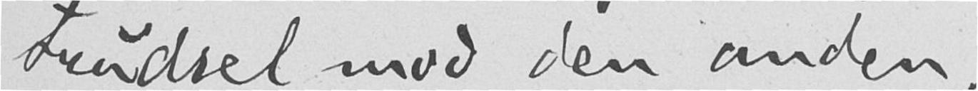trudsel mod den anden.
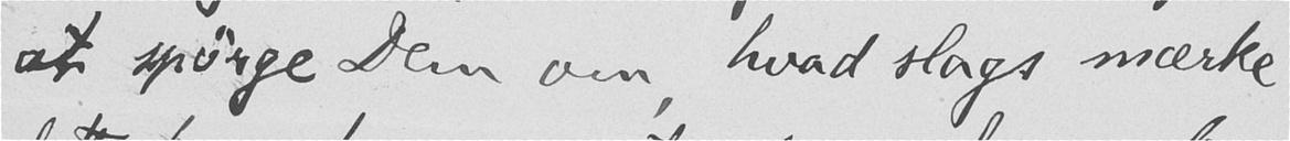at spørge Dem om, hvad slags mærke
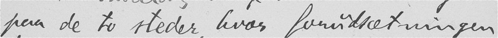paa de to steder, hvor forudsætningen
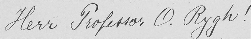Herr Professor O. Rygh!
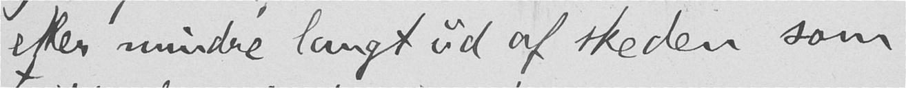eller mindre langt ud af skeden som
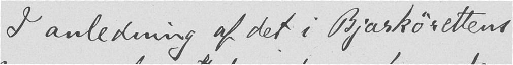I anledning af det i Bjarkørettens
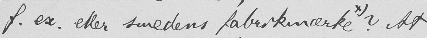f. ex. eller smedens fabrikmærke x)? At
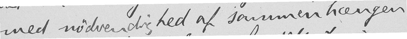med nødvendighed af sammenhængen
